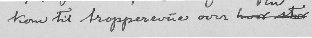kom til tropperevue over hvor stor
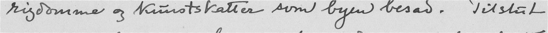rigdomme og kunstskatter som byen besad. Tilslut
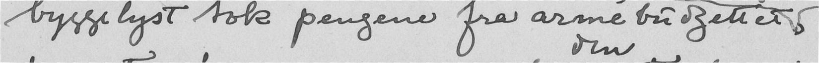byggelyst tok pengene fra armébudgettet
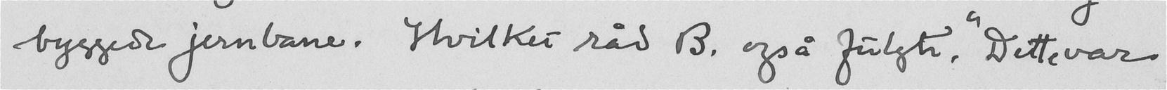byggede fernbane. Hvilket råd B. også fulgte. "Dette var
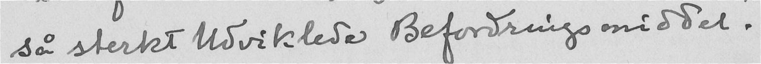så sterkt Udviklede Befordringsmiddel."
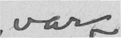var
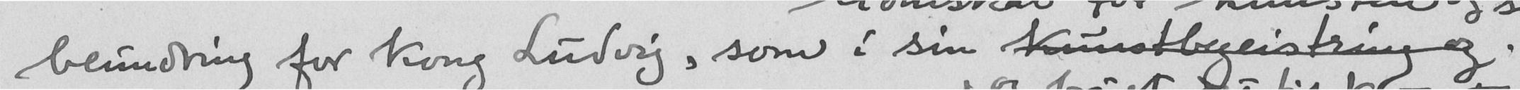beundring for kong Ludvig, som i sin kunstbegeistring og
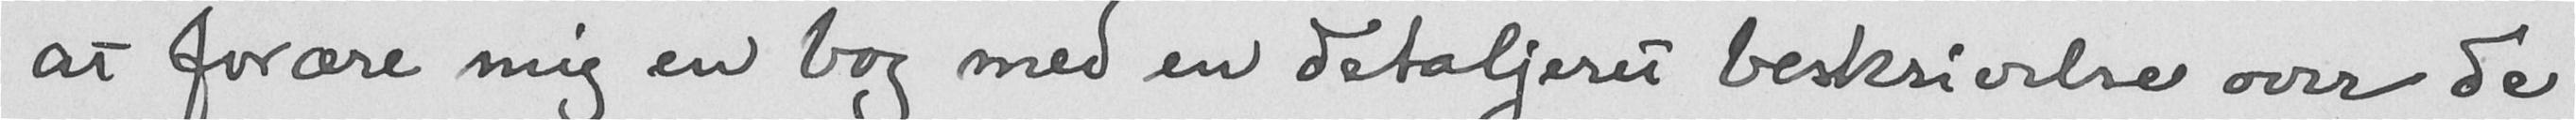at forære mig en bog med en detaljeret beskrivelse over de
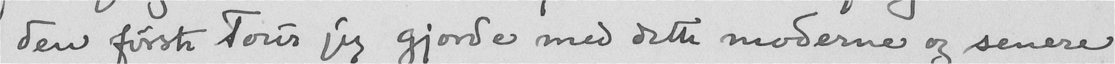den første Tour jeg gjorde med dette moderne og senere
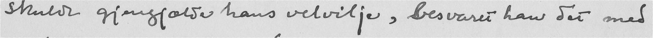skulde gjengjælde hans velvilje, besvaret han det med
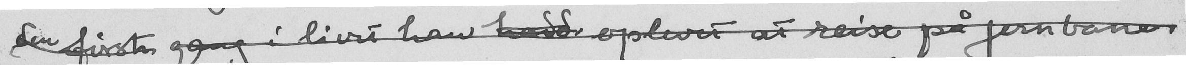den første gang i livet han hadd oplevet at reise på jernbane.
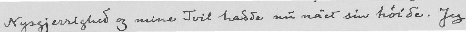Nysgjerrighed og mine Tvil hadde nu nået sin høide. Jeg
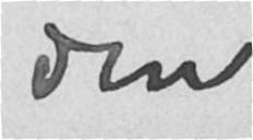den
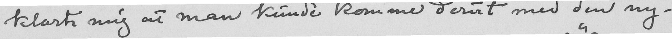klarte mig at man kunde komme derut med den ny-
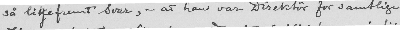så ligefremt svar, - at han var Direktør for samtlige
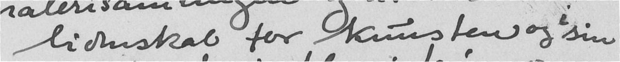lidenskab for kunsten og sin
